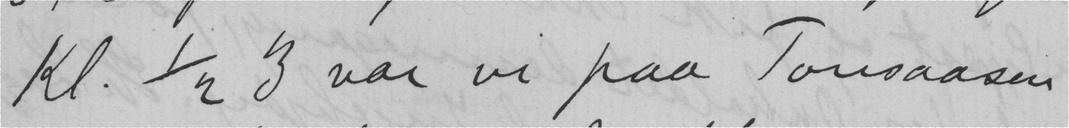Kl. 1/2 3 var vi paa Tonsaasen
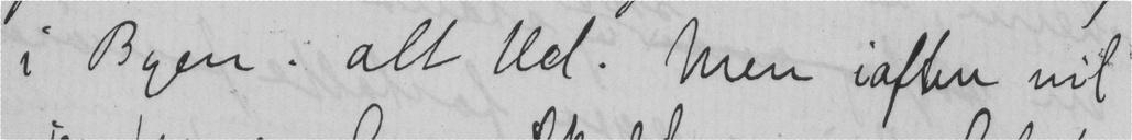i Byen. alt vel. Men iaften vil
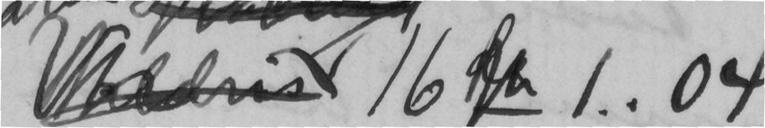16  1.04
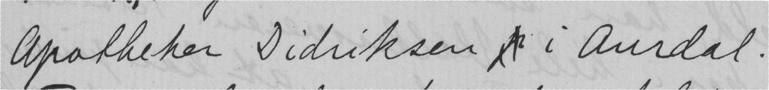Apotheker Didriksen  i Aurdal.
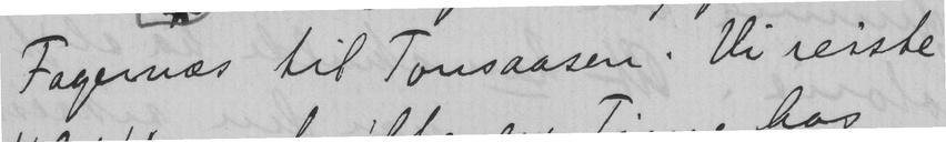Fagernæs til Tonsaasen. Vi reiste
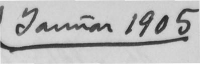Januar 1905
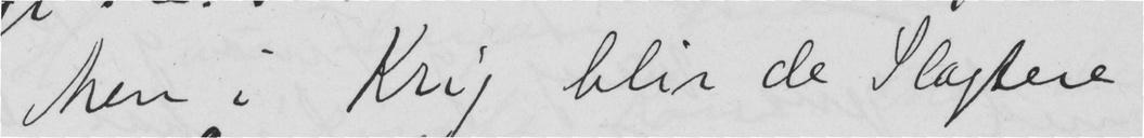Men i Krig blir de Slagtere
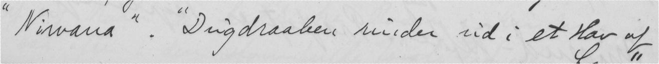"Nirvana". "Dugdraaben rinder ud i et Hav af
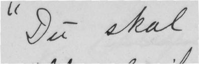"Du skal
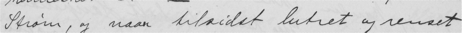Strøm, og naar tilsidst lutret og renset
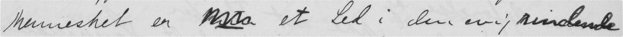Mennesket er  et Led i den evig rendende
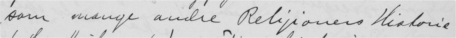som mange andre Religioners Historie
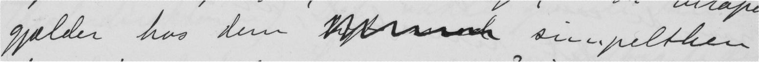gjælder hos dem  simpelthen
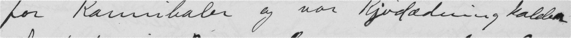for Kanibaler og vor kjødædning kalder
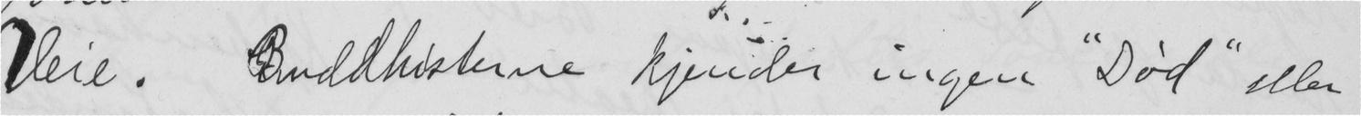Veie. Buddhisterne kjender ingen "Død" eller
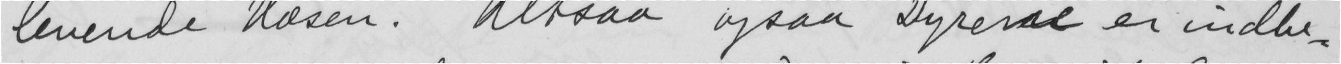levende Væsen". Altsaa ogsaa Dyreræt er indbe-
